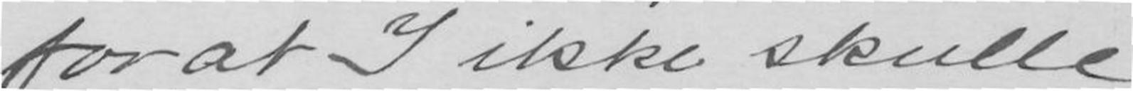for at I ikke skulle
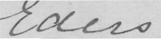Eders
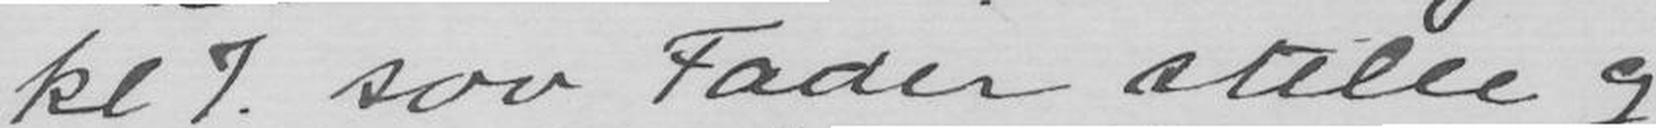kl 7. sov Fader stille og
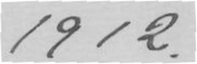1912.
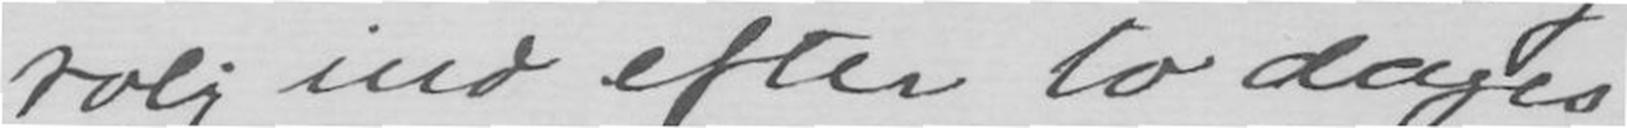rolig ind efter to dages
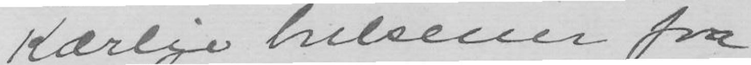Kærlige hilsener fra
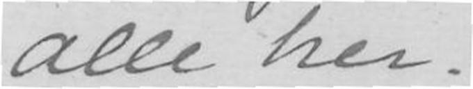Alle her
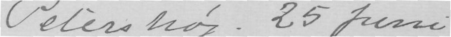Petershög. 25 Juni
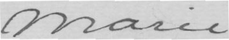Marie
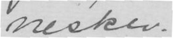nesket.
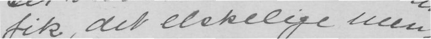fik, det elskelige men-
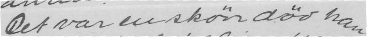Det var en skøn død han
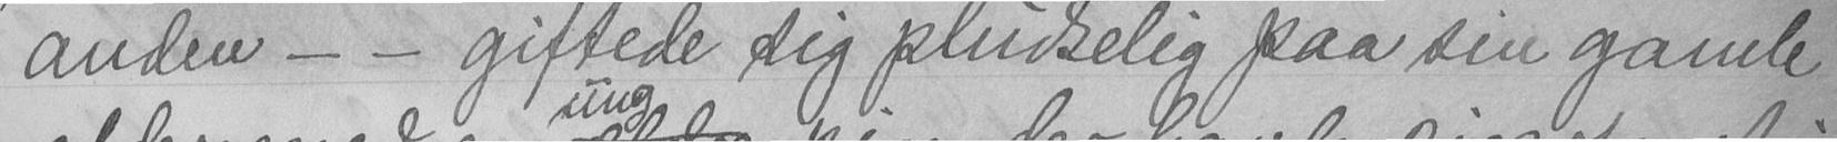anden - - giftede sig pludselig paa sin gamle
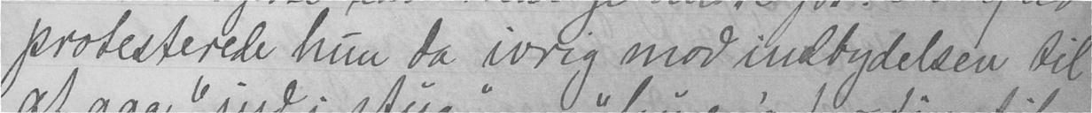protesterede hun da ivrig mod indbydelsen til
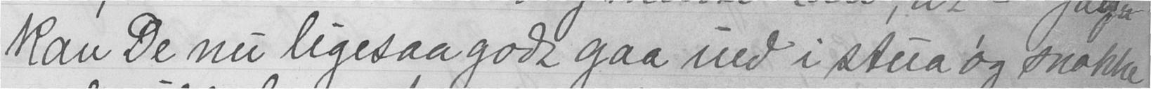ka De nu ligesaa godt gaa ind i stua og snakke
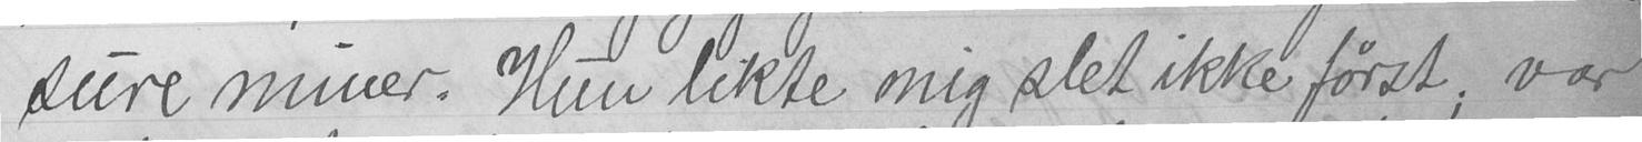sure miner. Hun likte mig slet ikke først; var
